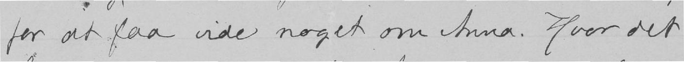for at faa vide noget om Anna. Hvor det
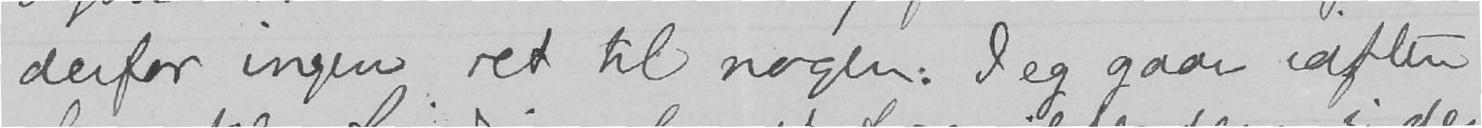derfor ingen ret til nogen. Jeg gaar iaften
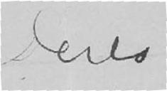Deres
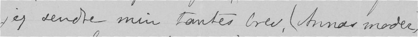jeg sendte min tantes brev, (Annas moder),
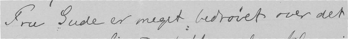Fru Gude er meget bedrøvet over det
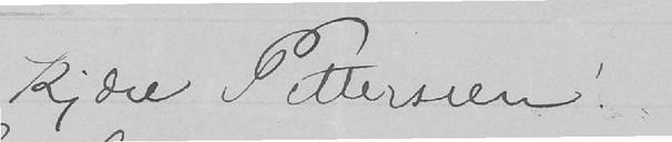Kjære Petterssen!
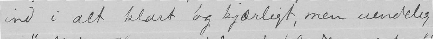ind i alt klart og kjærligt, men uendelig
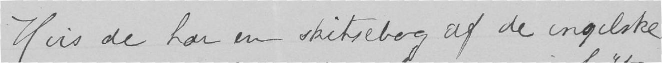Hvis de har en skitsebog af de engelske
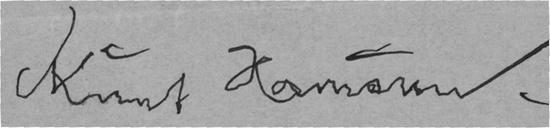Knut Hamsun.
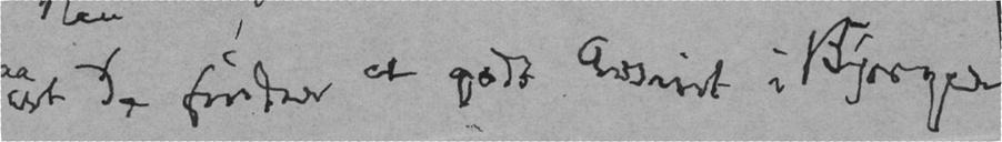at De finder et godt Avsnit i Bjørger
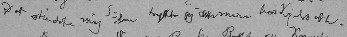Det stødte mig Siden trykte jeg ikke mere hos Kjelseth.
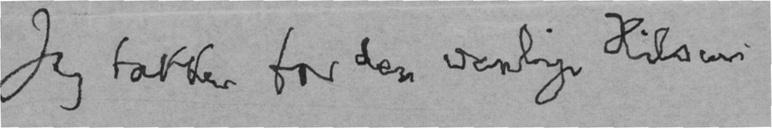Jeg takker for den venlige Hilsen.
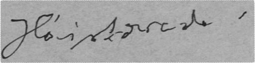Høistærede,
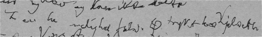Te en he melighet fulde Ø trykt hos Kjelseth
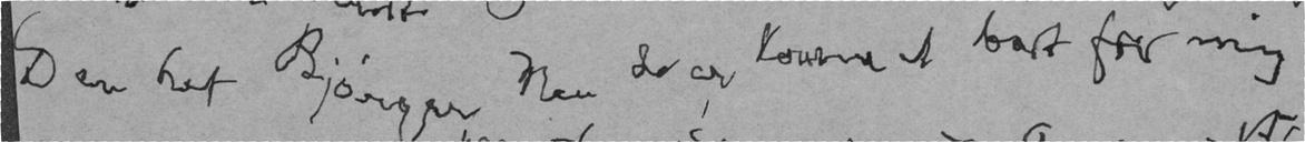Den het Bjørger Men de er kommet bort for mig
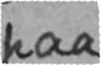paa
